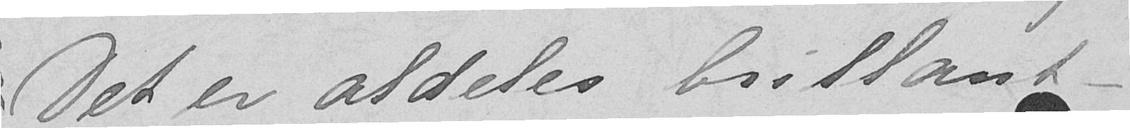Det er aldeles brillant -
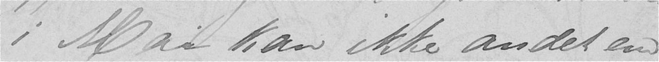i Mai kan ikke andet end
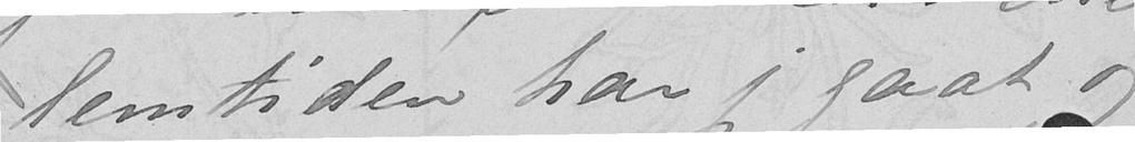lemtiden har jeg gaat og
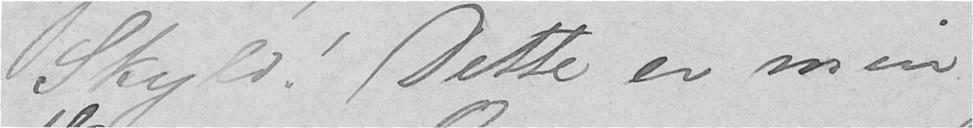Skyld! Dette er min
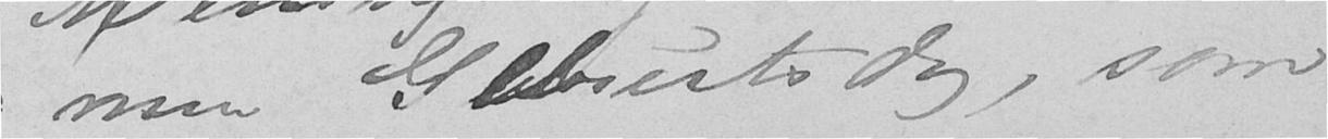min Geburtsdag, som
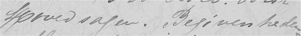Hovedsagen. Begivenheden
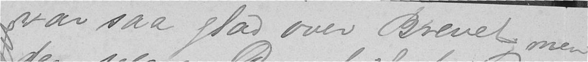var saa glad over Brevet, men
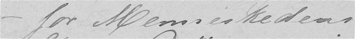- for Menneskedens
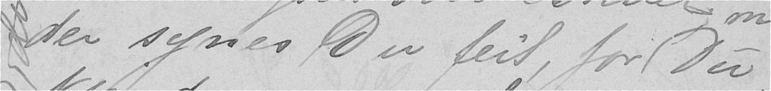der synes Du feil, for Du
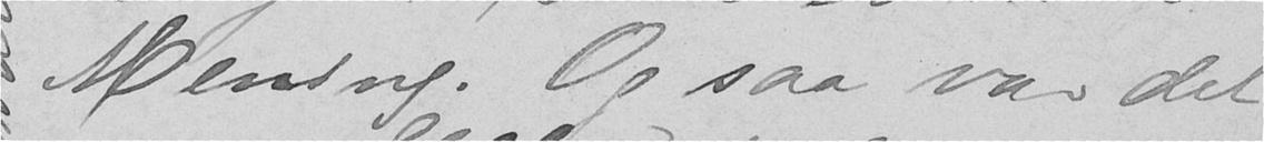Mening. Og saa var det
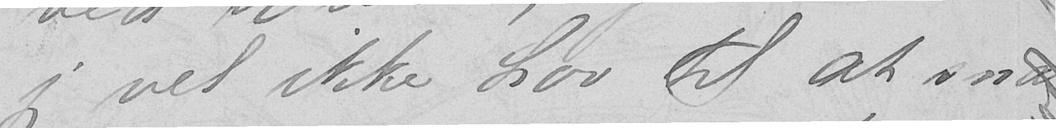jeg vel ikke Lov til at snak-
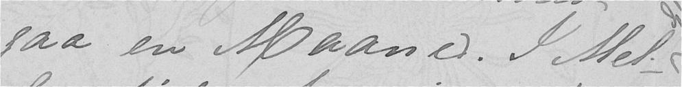gaa en Maaned. I Mel-
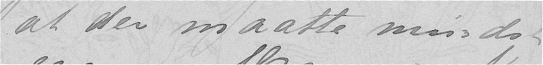at der maatte mindst
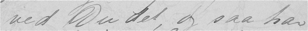ved Du det, og saa har
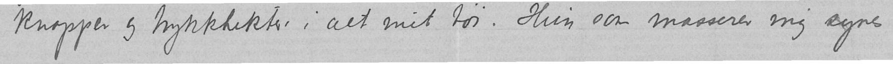knapper og trykkhekter i alt mit tøi. Hun som masserer mig synes
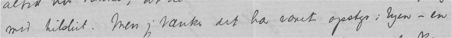med tilslut. Men jeg tænker det har været opstyr i byen - en
